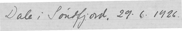Dale i Søndfjord. 29.6.1926.
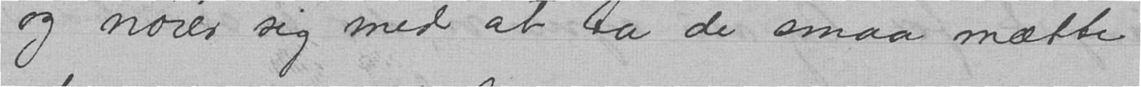og nøier sig med at ta de smaa mætte
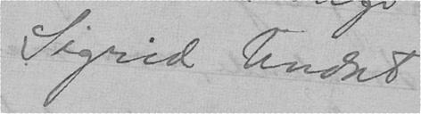Sigrid Undset
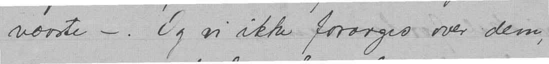værste -. Og vi ikke forarges over dem,
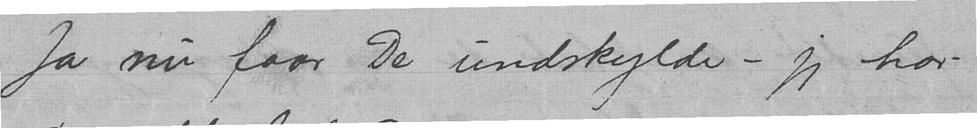Ja nu faar De undskylde - jeg har
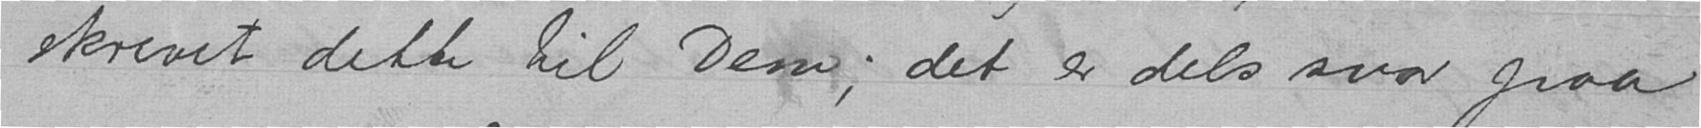skrevet dette til Dem; det er dels svar paa
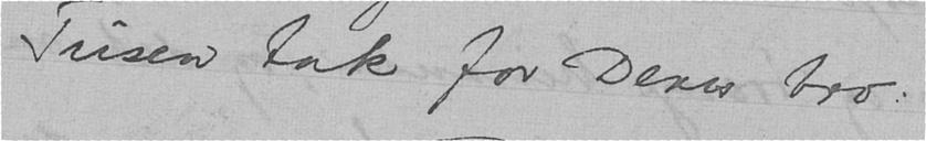Tusen tak for Deres brev.
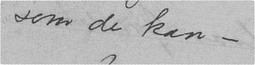som de kan -
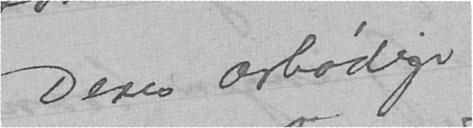Deres ærbødige
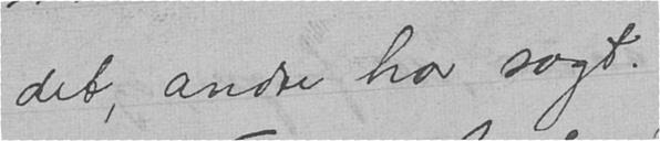det, andre har sagt.
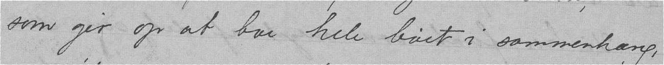som gir op at leve hele livet i sammenhæng,
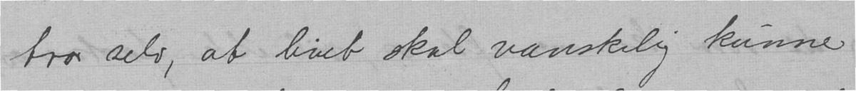tro selv, at livet skal vanskelig kunne
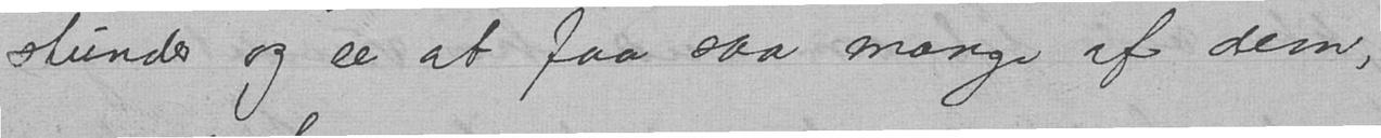stunder og se at faa saa mange af dem,
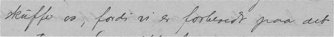skuffe os, fordi vi er forberedt paa det
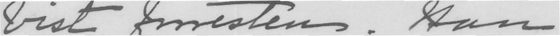Vist forresten. Han
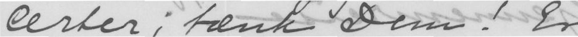serter, tænk Dem! Er
